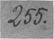255.
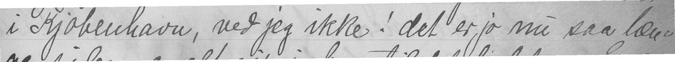i Kjøbenhavn, ved jeg ikke! det er jo nu saa læn-
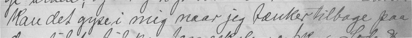kan det gyse i mig naar jeg tænker tilbage paa
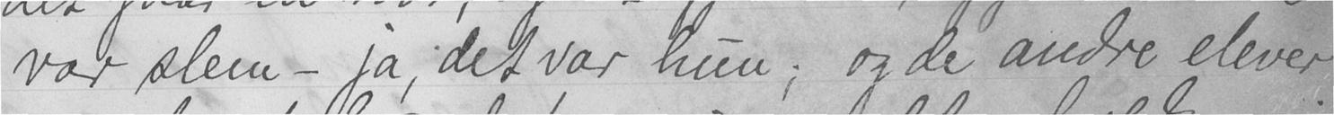var slem - ja, det var hun; og de andre elever
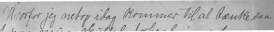Hvorfor jeg netop idag kommer til at tænke saa
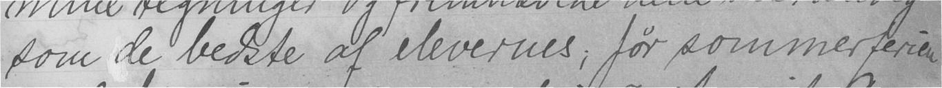som de bedste af elevernes; før sommerferien
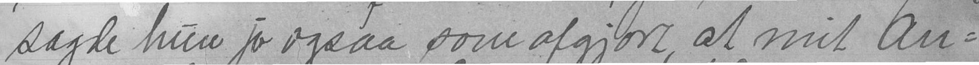sagde hun jo ogsaa som afgjort, at mit an-
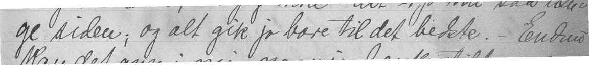ge siden; og alt gik jo bare til det bedste. - Endnu
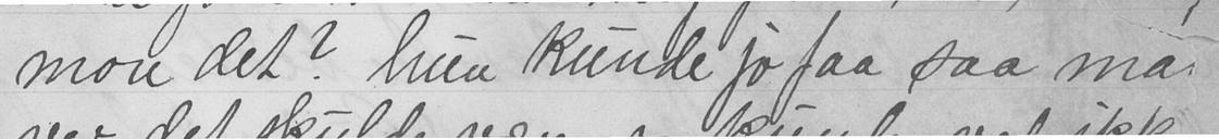mon det? hun kunde jo faa saa ma
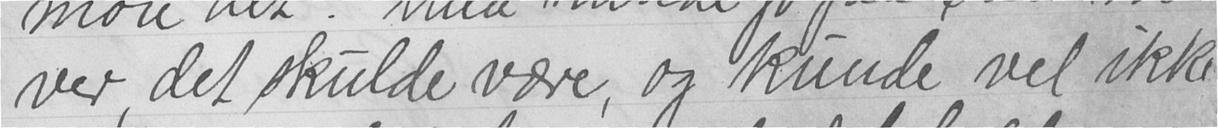ver, det skulde være, og kunde vel ikke
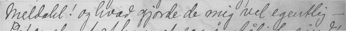Meldahl! og hvad gjorde de mig vel egentlig -
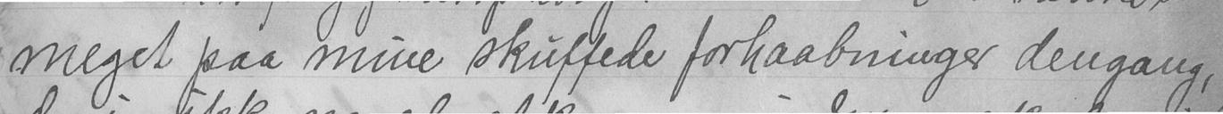meget paa mine skuffede forhaabninger dengang,
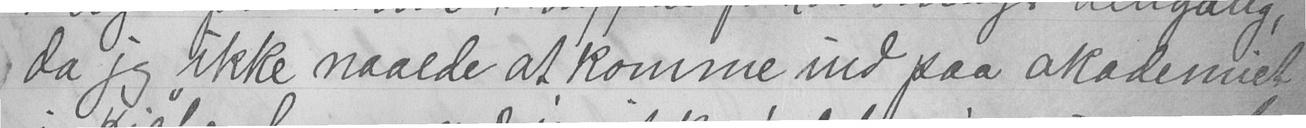da jeg ikke naaede at komme ind paa akademiet
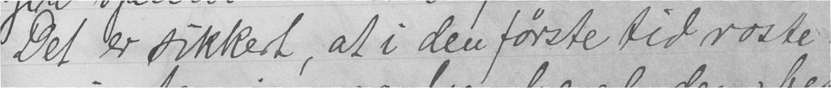"Det er sikkert, at i den første tid roste
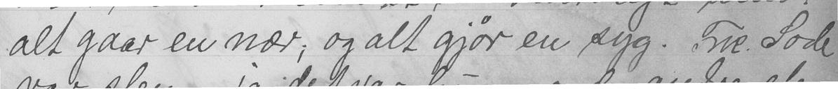alt gaar en nær; og alt gjør en syg. Frk. Sode
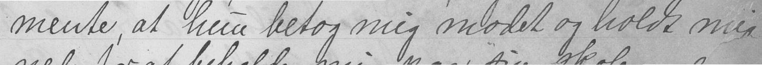mente, at hun betog mig modet og holdt mig
